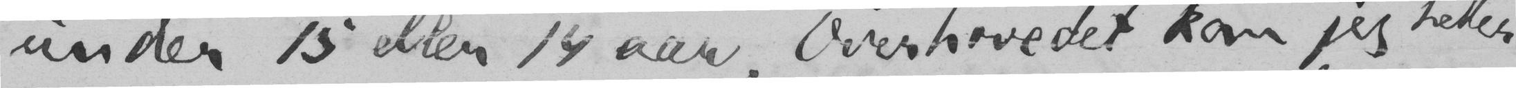under 15 eller 14 aar. Overhovedet kan jeg heller
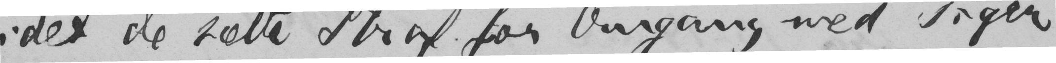idet de sætte Straf for omgang med Piger
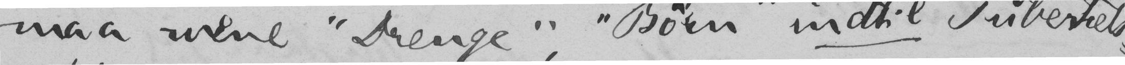maa mene «Drenge», «børn» indtil Pubertets-
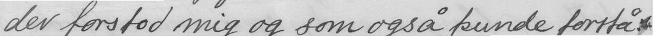der forstod mig og som også kunde forstå
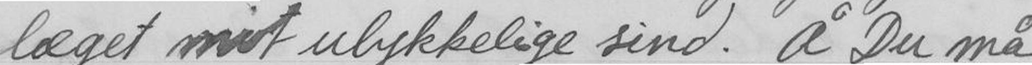læget mit ulykkelige sind. Å Du må
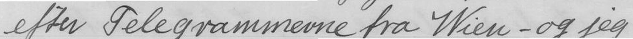efter Telegrammerne fra Wien - og jeg
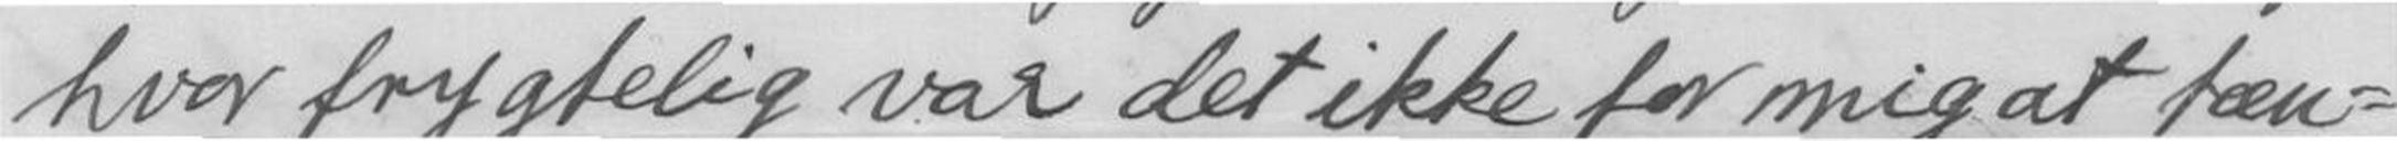hvor frygtelig var det ikke for mig at tæn-
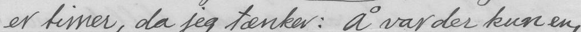er timer, da jeg tænker: Å var der kun en,
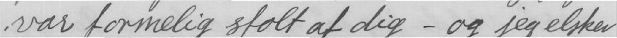var formelig stolt af dig - og jeg elsker
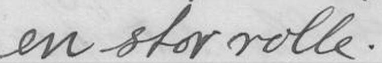en stor rolle.
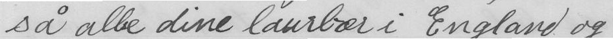så alle dine laurbær i England og
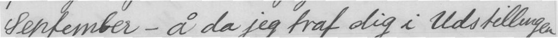September - å da jeg traf dig i Udstillingen
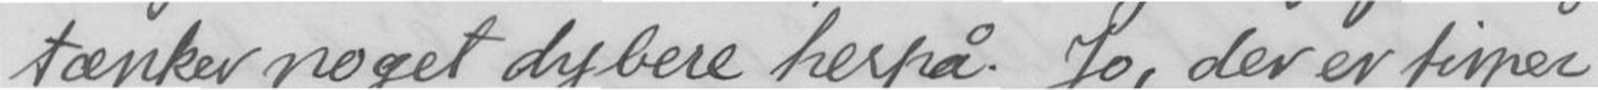tænker noget dybere herpå. Jo, der er timer
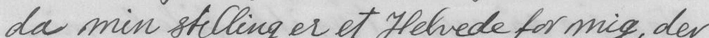da min stilling er et Helvede for mig, der
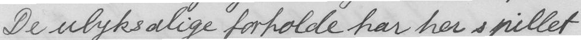De ulyksalige forholde har her spillet
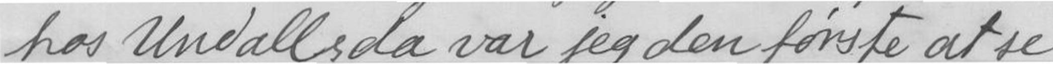hos Undalls da var jeg den første at se
